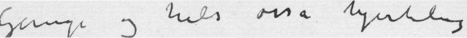Gange og hils osså hjertelig
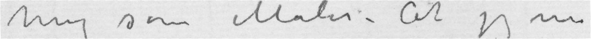mig som Maler – At jeg nu
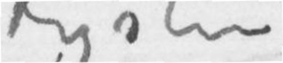Kysten
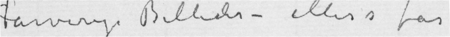farverige Billeder – ellers får
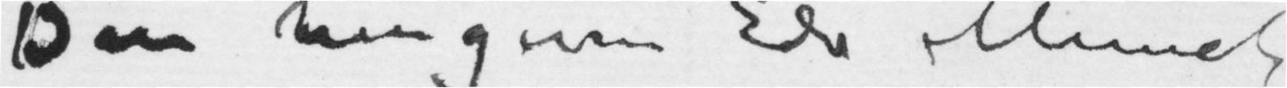Din hengivne Edv Munch
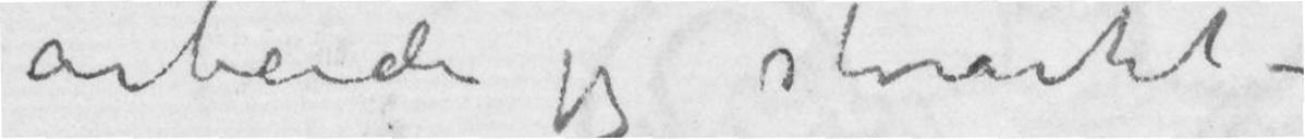arbeider jeg storartet –
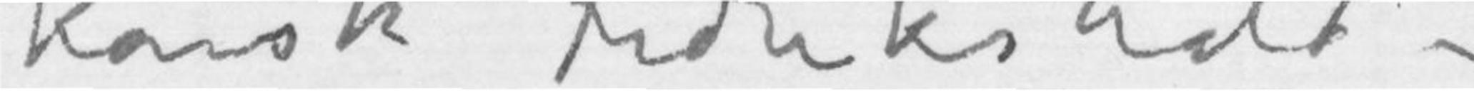Kanskje Fredrikshald –
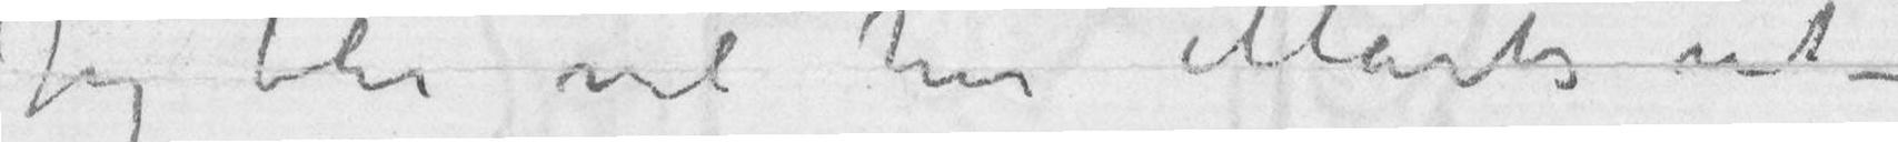Jeg blir vel her Marts ut –
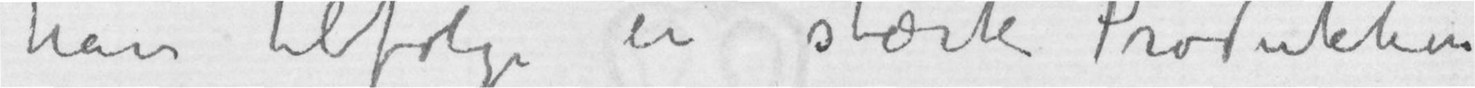have tilfølge en stærk Produktion
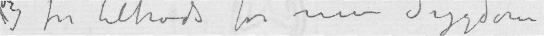og for tiltrods for min Sygdom
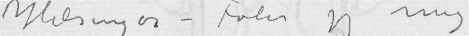Helsingør – Føler jeg mig
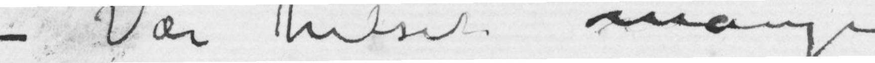– Vær hilset mange
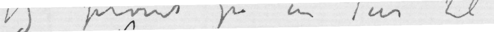jeg prøvet på en Tur til
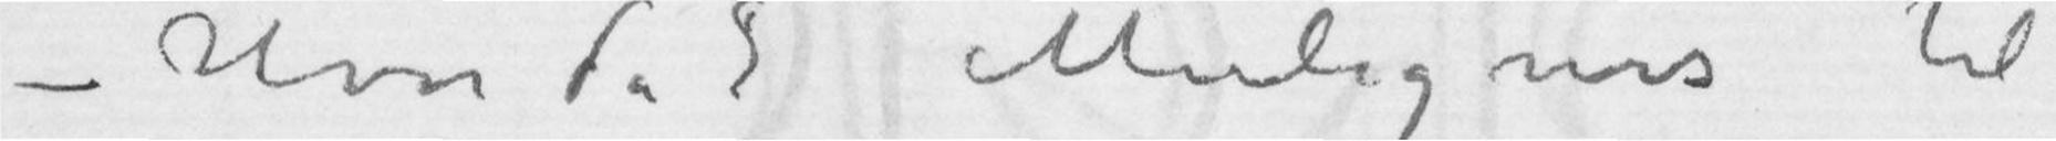– Hvor da? Muligens til
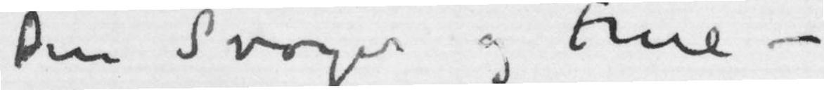din Svoger og Frue –
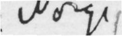Norge
